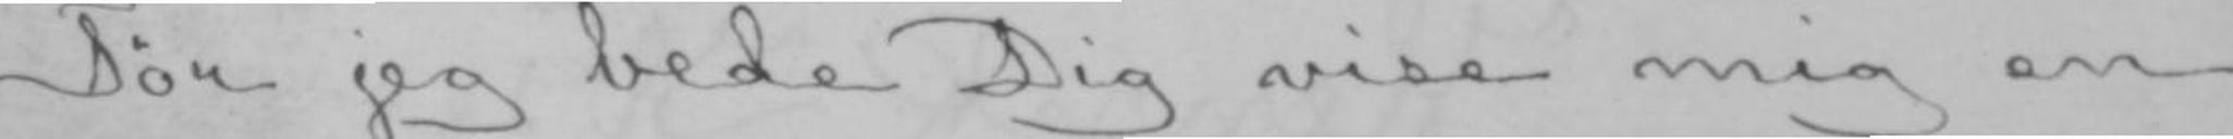Tør jeg bede Dig vise mig en
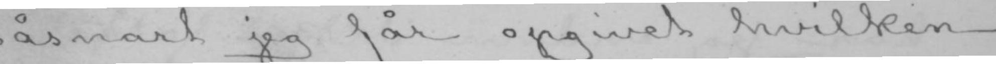såsnart jeg får opgivet hvilken
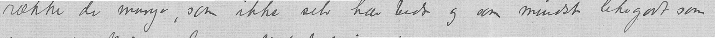række de mange, som ikke selv har bedt og som mindst likegodt som
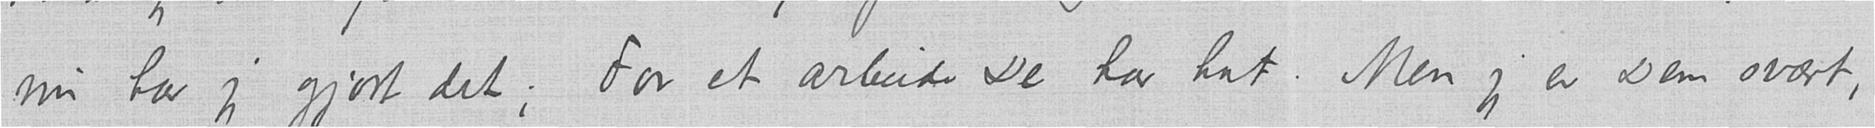nu har jeg gjort det; For et arbeide De har hat. Men jeg er Dem svært,
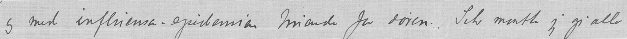og med influensa-epidemien truende for døren. Selv maatte jeg gi alle
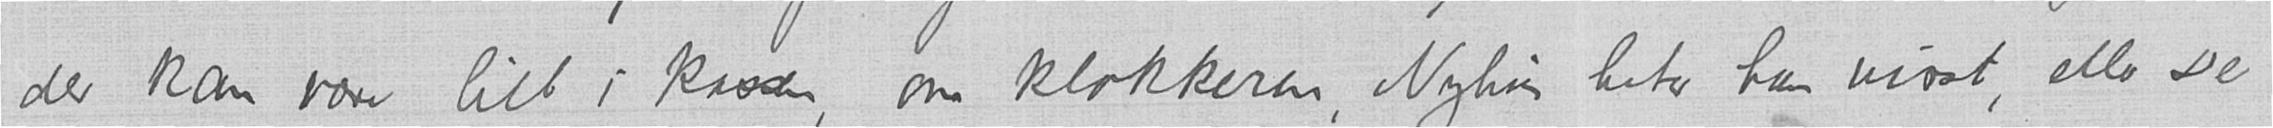der kan være litt i kassen, om klokkeren, Nyhus heter han visst, eller De
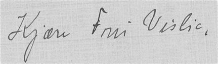Kjære Fru Vislie,
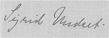Sigrid Undset.
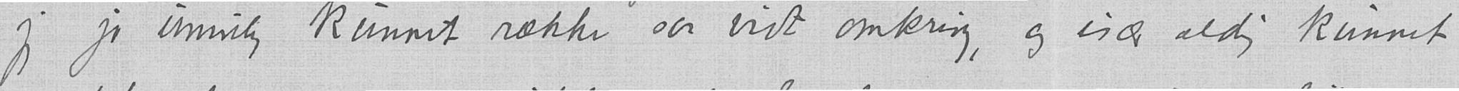jeg jo umulig kunnet række saa vidt omkring, og især aldrig kunnet
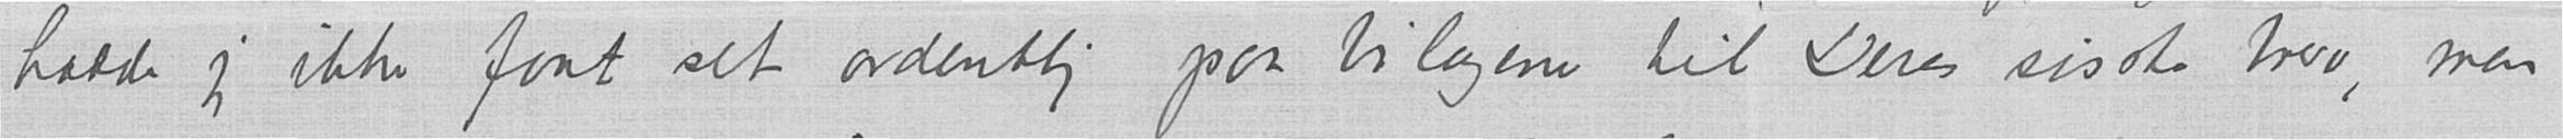hadde jeg ikke faat set ordentlig paa bilagene til Deres sisste brev, men
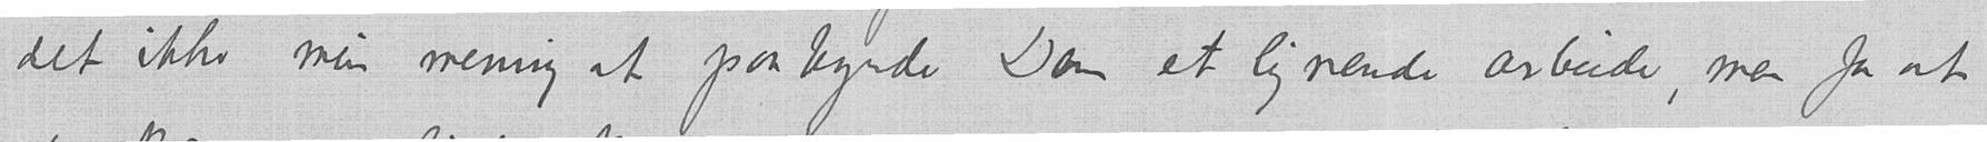det ikke min mening at paaskynde Dem et lignende arbeide, men for at
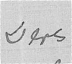Deres
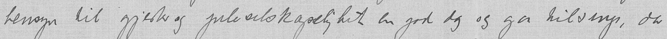hensyn til gjester og juleselskapelighet en god dag og gaa tilsengs, da
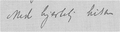Med hjertelig hilsen
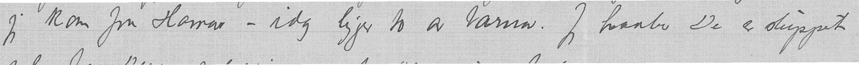jeg kom fra Hamar – idag ligger to av barna. Jeg haaber De er sluppet
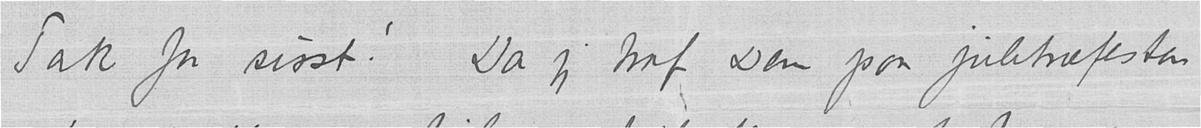Tak for sisst! Da jeg traf Dem paa juletræfesten
